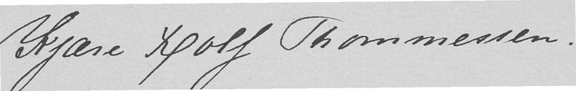Kjære Rolf Thommessen.
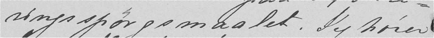ringsspørgsmaalet. Jeg hører
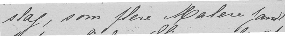slag, som flere Malere fandt
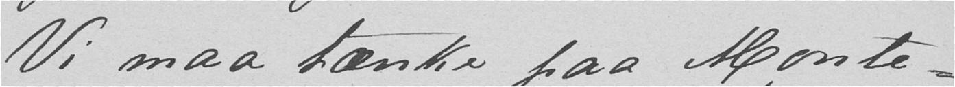Vi maa tænke paa Monte-
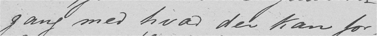gang med hvad der kan for-
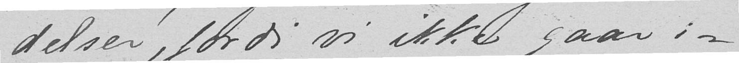delser, fordi vi ikke gaar i-
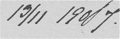13/11 1907
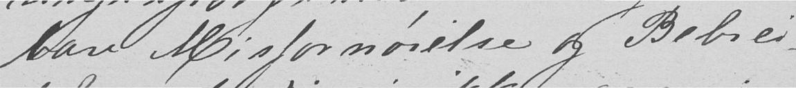bare Misfornøielse og Bebrei-
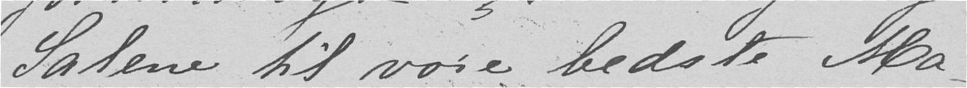Salene til vore bedste Ma-
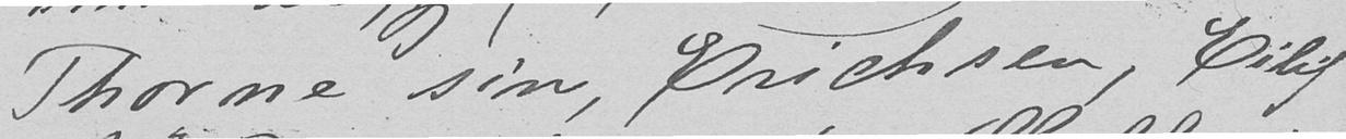Thorne sin, Erichsen, Eilif
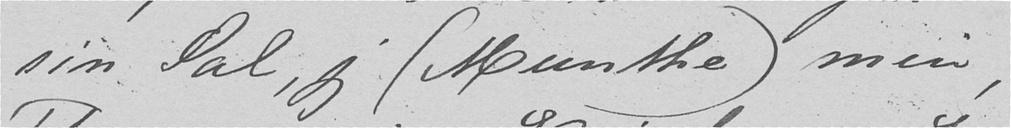sin Sal, jeg (Munthe) min,
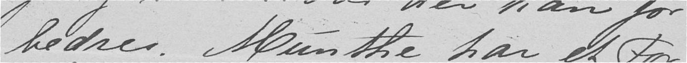bedres. Munthe har et For-
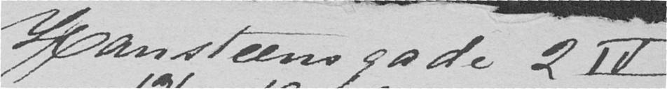Hansteensgade 2 IV
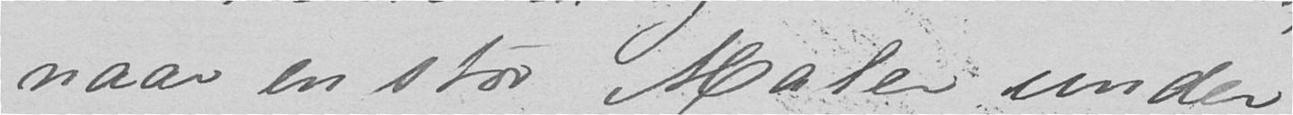naar en stor Maler under
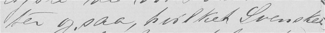ter ogsaa, hvilket Svensker-
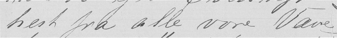hest fra alle vore Væve-
